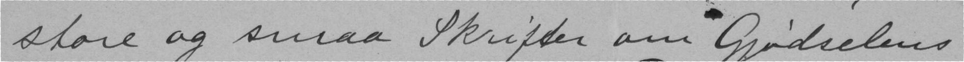store og smaa Skrifter om Gjødselens
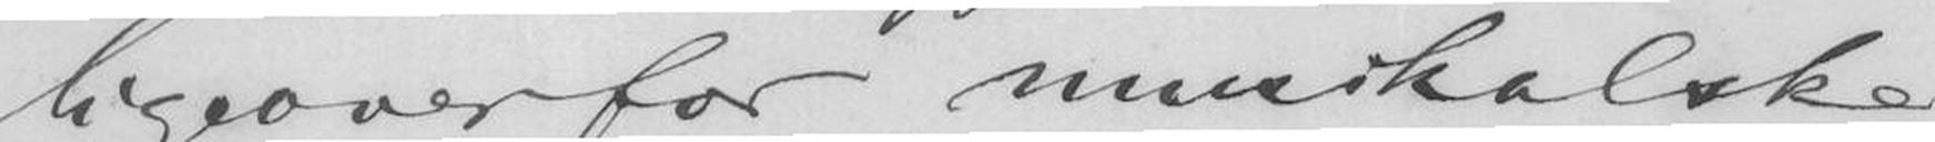ligeoverfor musikalske
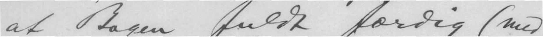at Bogen fuldt Færdig
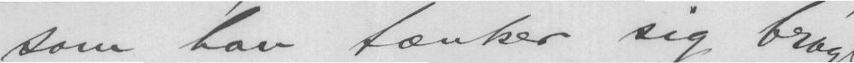som han tænker sig bragt
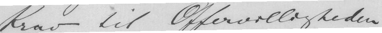Krav til Offervilligheden
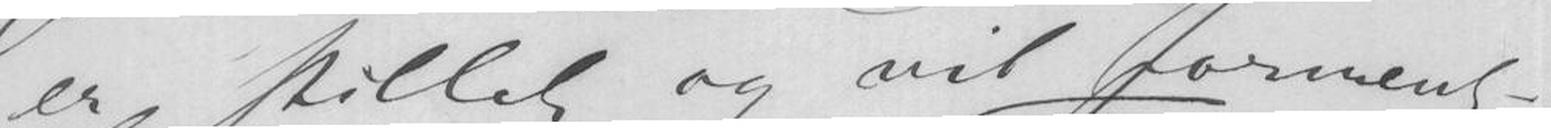er stillet og vil forment-
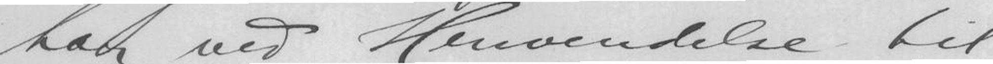har ved Henvendelse til
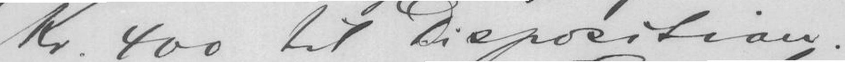Kr.400 til Disposition.
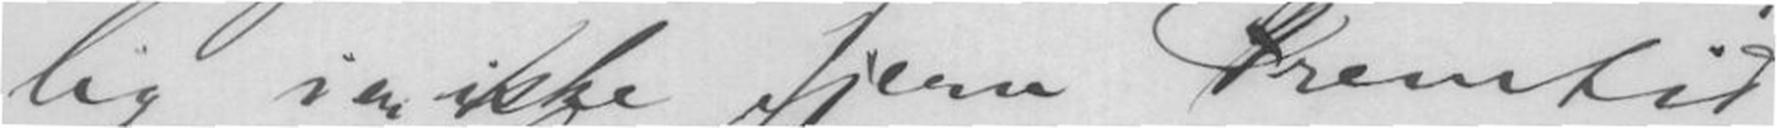lig i en ikke fjern Fremtid
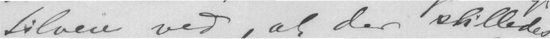tilveie ved, at der stilledes
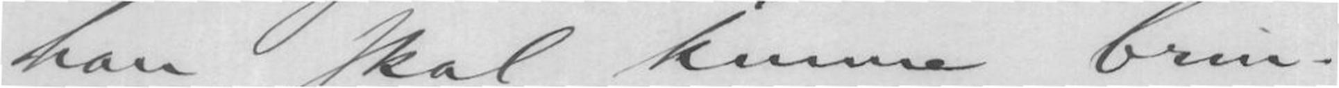han skal kunne brin-
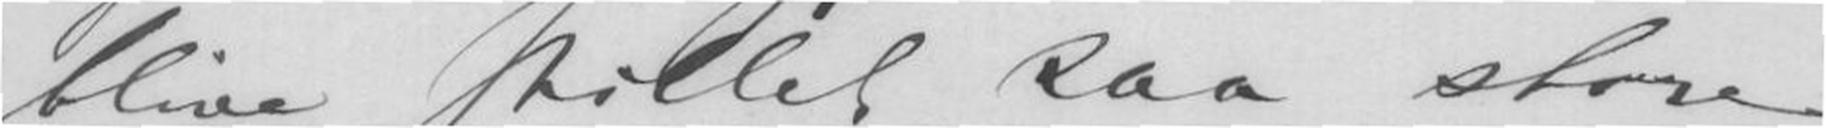blive stillet saa store
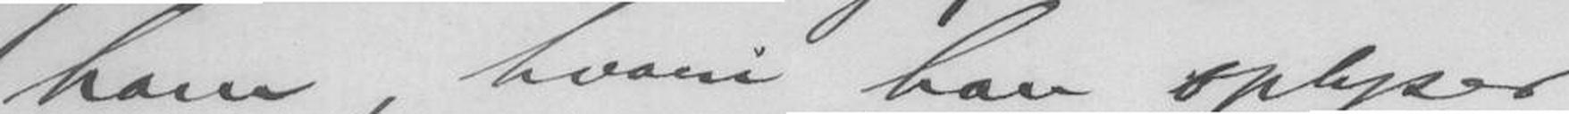ham, hvori han oplyser
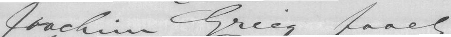Joachim Grieg faaet
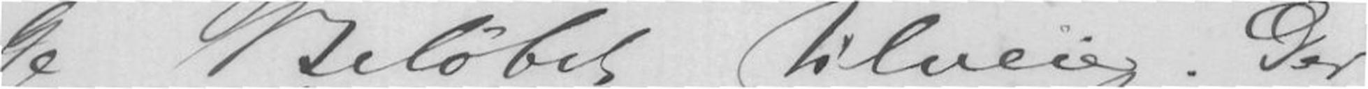ge Beløbet tilveie. Der
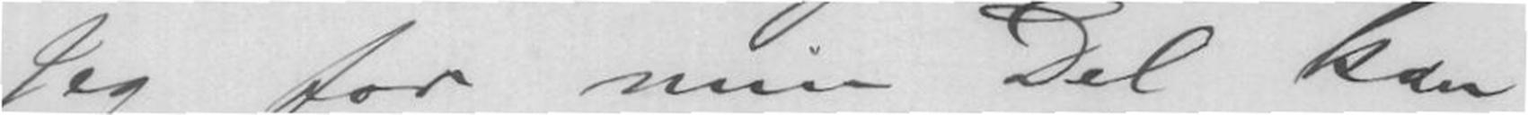Jeg for min Del kan
